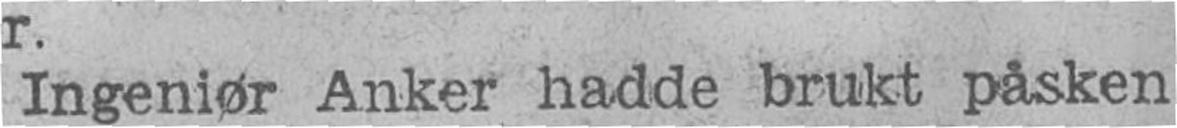Ingeniør Anker hadde brukt påsken
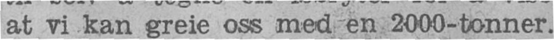at vi kan greie oss med en 2000-tonner.
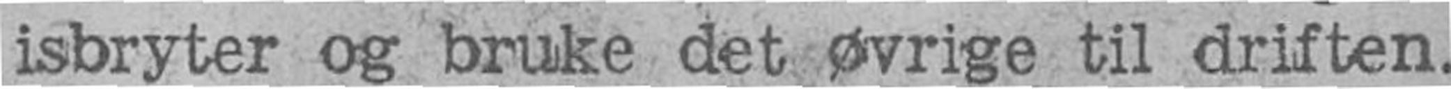isbryter og bruke det øvrige til driften.
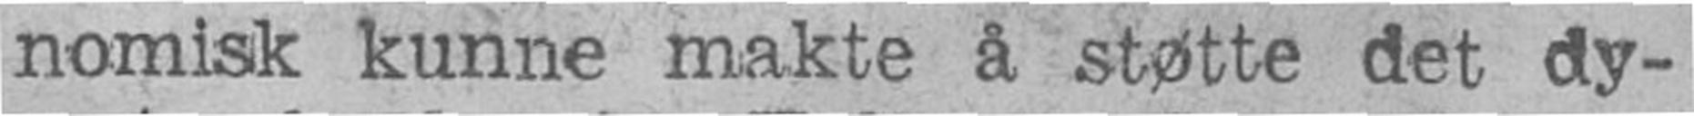nomisk kunne makte å støtte det dy-
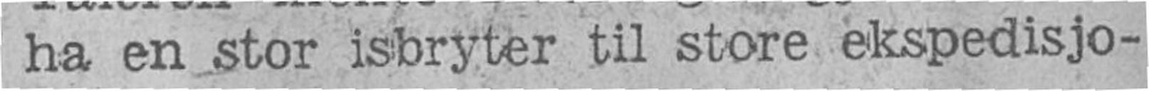ha en stor isbryter til store ekspedisjo-
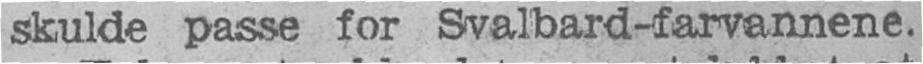skulde passe for Svalbard-farvannene.
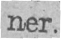ner.
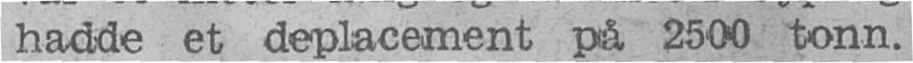hadde et deplacement på 2500 tonn.
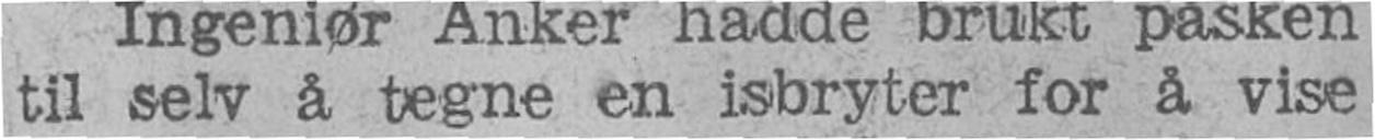til selv å tegne en isbryter for å vise
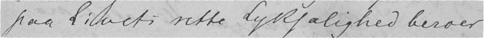paa Livets rette Lyksalighed beroer
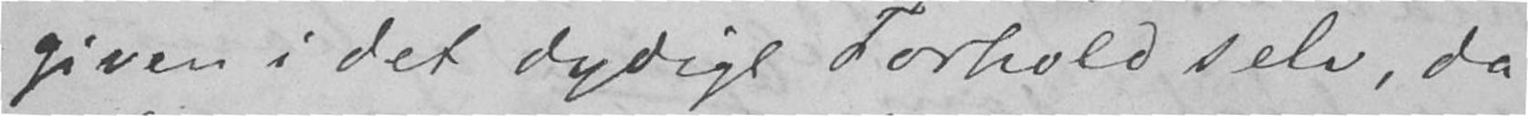given i det dydige Forhold selv, da
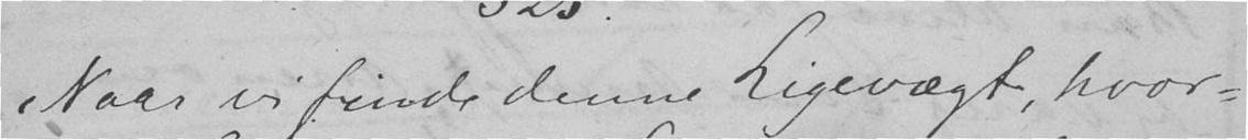Naar vi finde denne Ligevægt, hvor-
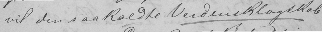vil den saakaldte Verdensklogskab
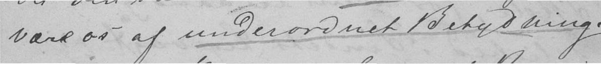være os af underordnet Betydning.
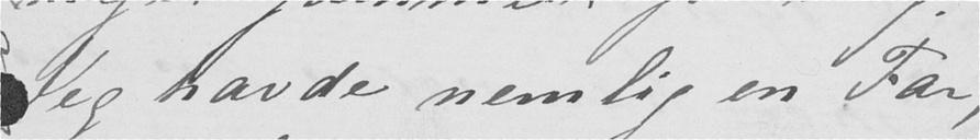Jeg havde nemlig en Far,
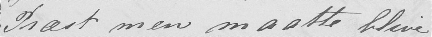Præst men maatte blive
